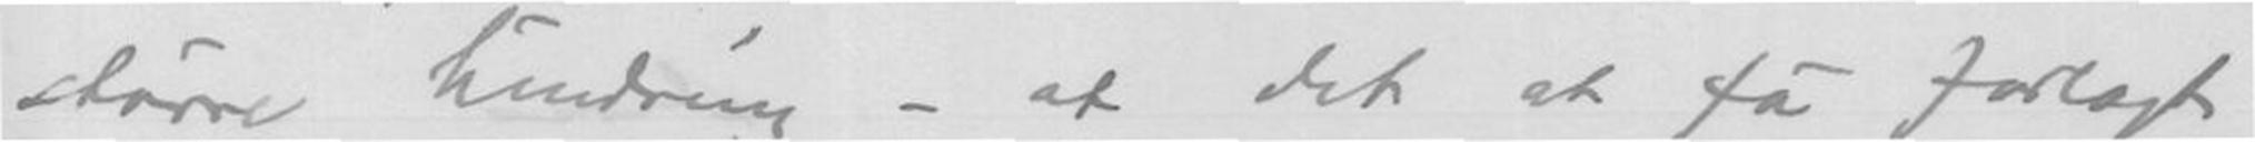større hindring - at det at få forlagt
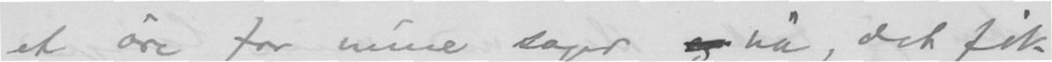et øre for mine sager og nå, det fik
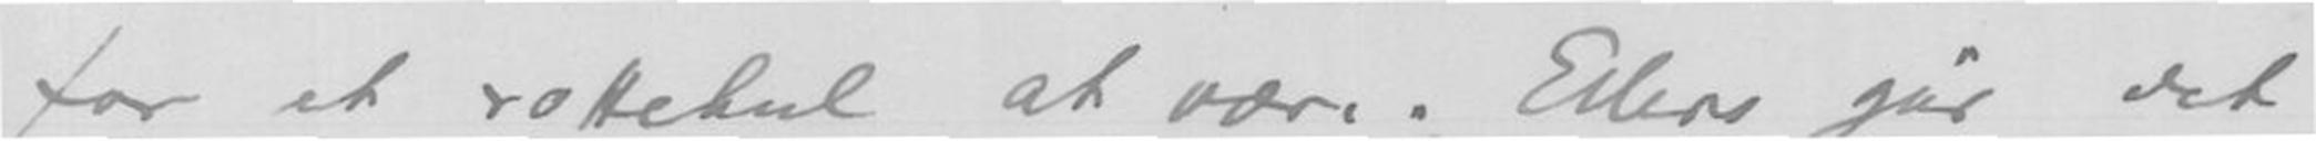for et rottehul at være. Ellers går det
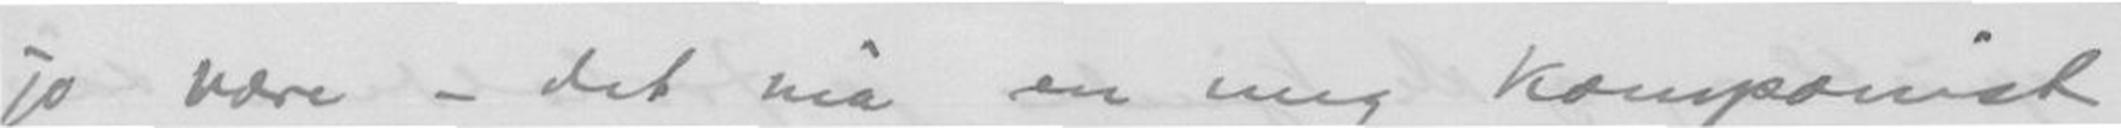jo være - det må en ung komponist
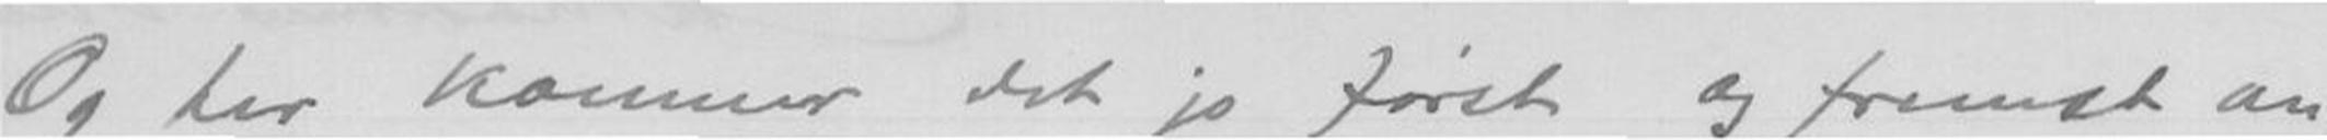Og her komme det jo først og fremst an
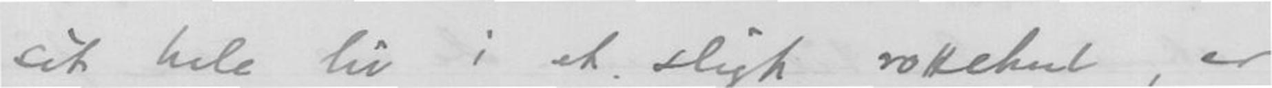sit hele liv i et sligt rottehul, er
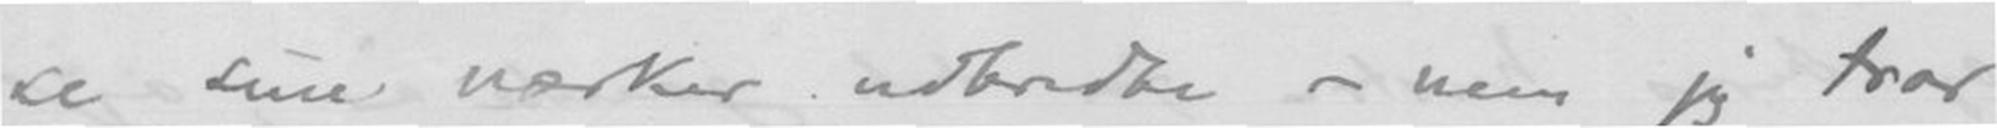se sine værker udbredte - men jeg tror
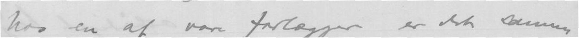hos en af vore forlæggere er det samme
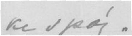ke spøg.
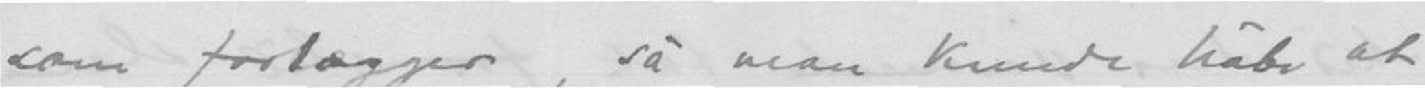som forlægger, så man kunde håbe at
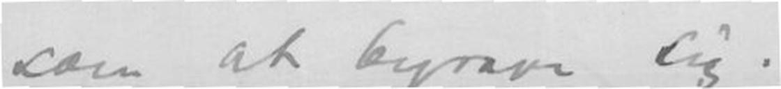som at begrave sig.
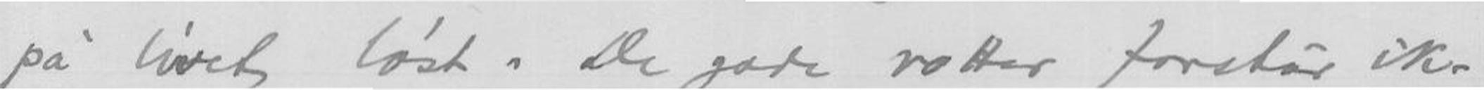på livet løst. De gode rotte forstår ik-
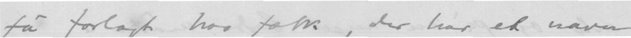få forlagt hos folk, der har et navn
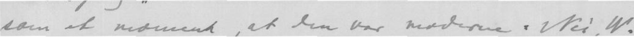som et moment, at den var moderne. Nei, W.
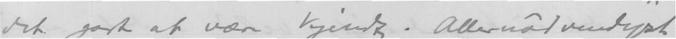det godt at være kjendt. Allernødvendigst
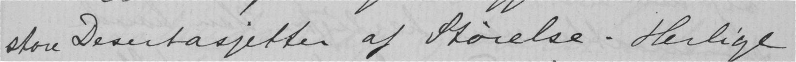store Desertasjetter af Størelse. Herlige
